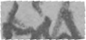til
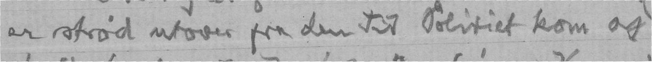er strød utover fra den Tid Politiet kom og
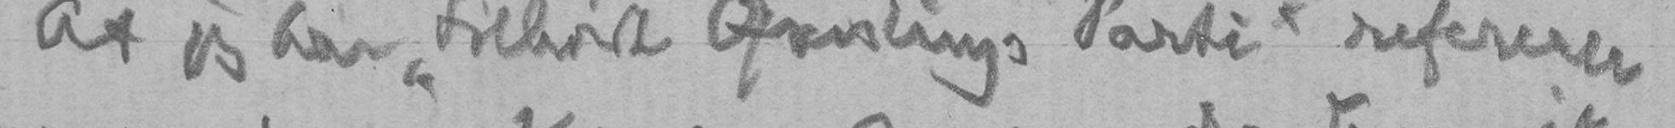At jeg har "tilhørt Quislings Parti" refererer
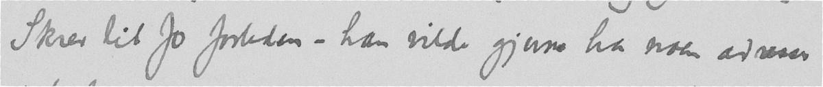Skrev til Jo forleden – han vilde gjerne ha noen adresser
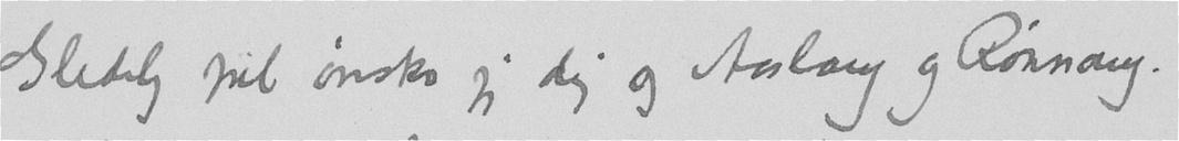Gledelig jul ønsker jeg dig og Aaslaug og Rønnaug.
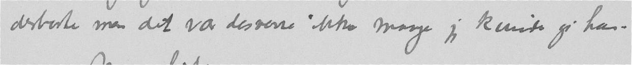derborte men det var desverre ikke mange jeg kunde gi ham -
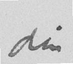din
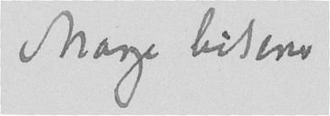Mange hilsener
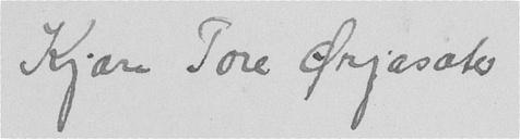Kjære Tore Ørjasæter
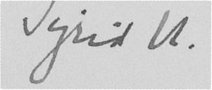Sigrid U.
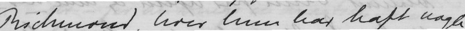Richmond, hvor hun har haft nogle
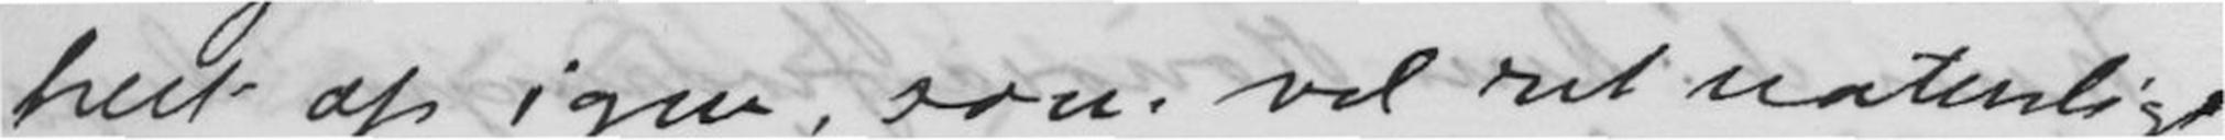helt op igen, som vel ret naturligt
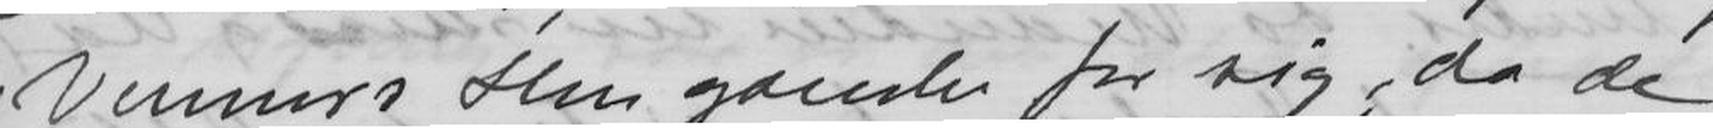Venners Hem ganske for sig, da de
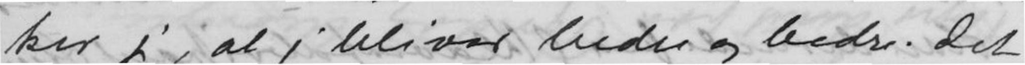ker jeg, at j bliver bedre og bedre. Det
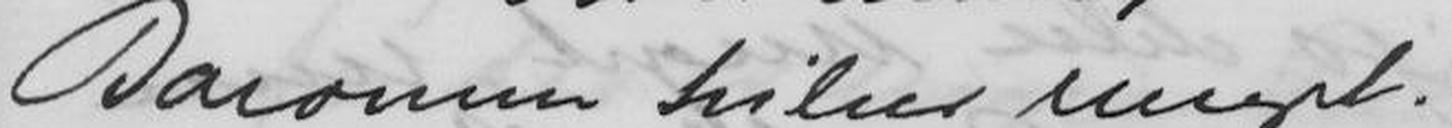Baronen hilser meget.
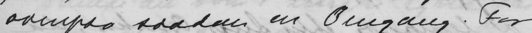ovenpaa saadan en Omgang. For
